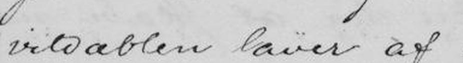vildæblen laver af
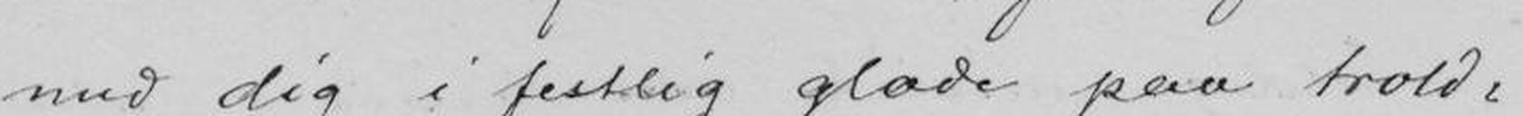med dig i festlig glæde paa trold-
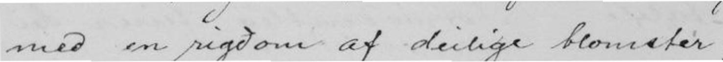med en rigdom af deilige blomster
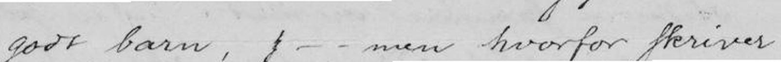godt barn, - men hvorfor skriver
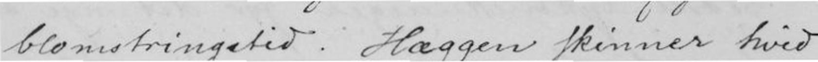blomstringstid. Hæggen skinner hvid
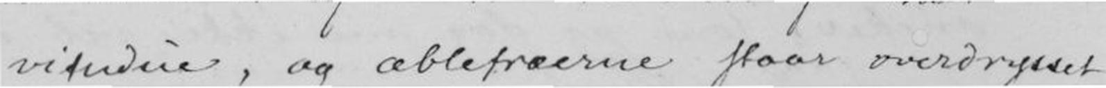vindue, og æbletræerne staar overdrysset
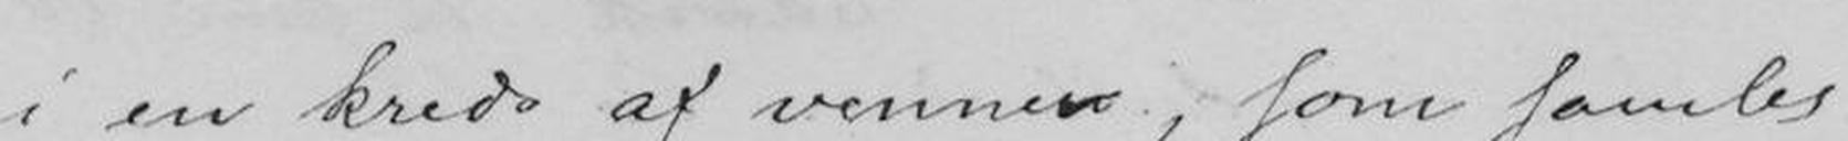i en kreds af venner, som samles
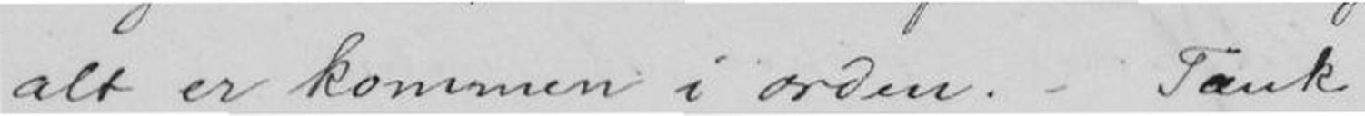alt er kommen i orden. - Tænk
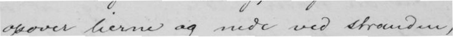opover lierne og nede ved stranden,
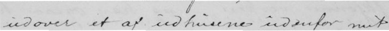udover et af udhusene udenfor mit
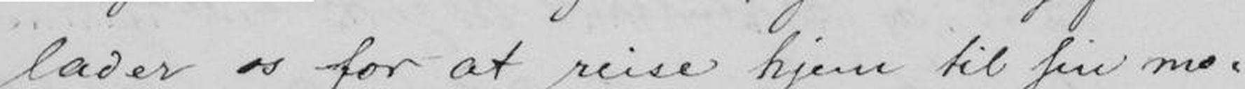lader os for at reise hjem til sin mo-
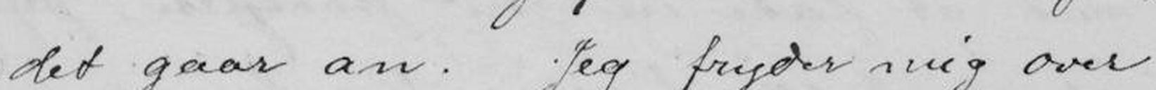det gaar an. Jeg fryder mig over
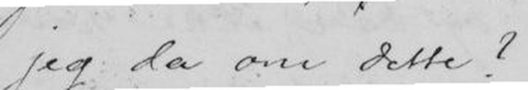jeg da om dette?
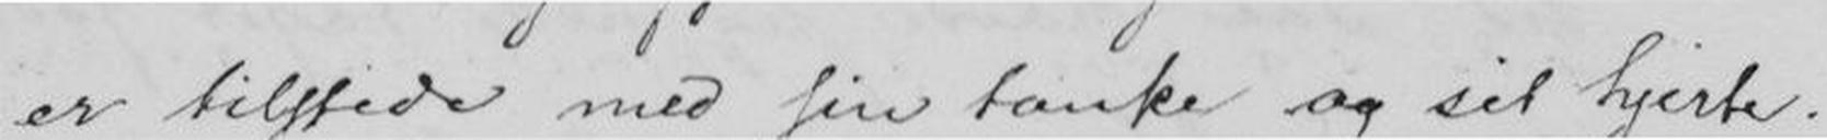er tilstede med sin tanke og sit hjerte.
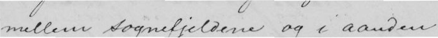mellem sognefjeldene og i aanden
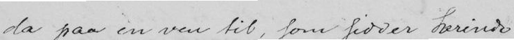da paa en ven til, som sidder herinde
